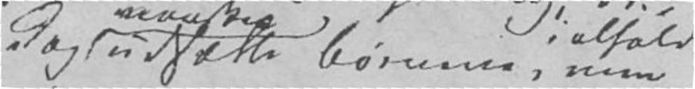Dog maaskee udsætte Børnene, men
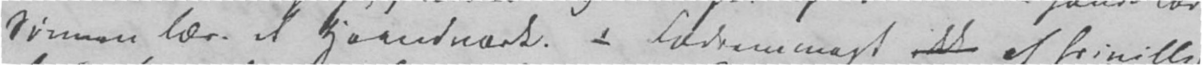Sønnen lære et Haandværk. – Fædrenemagt ikke af frivillig
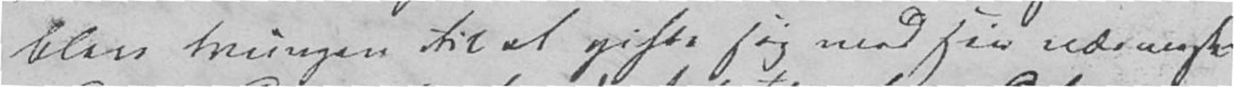blev tvungen til at gifte sig med sin nærmeste
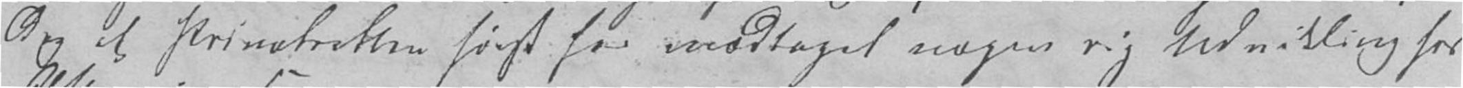dog at Privatretten først har modtaget nogen rig Udvikling hos
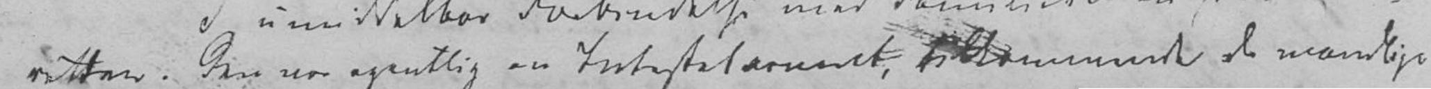retten. Den var egentlig en Intestatarveret, tilkommende de mandlige
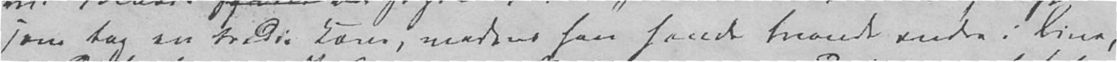som tog en tredie Kone, medens han havde tvende andre i Live,
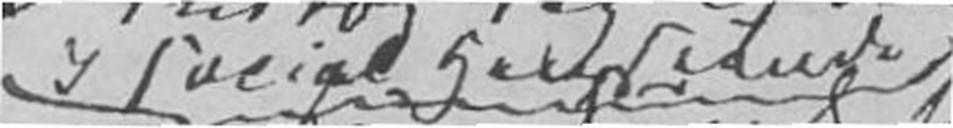i social Henseende
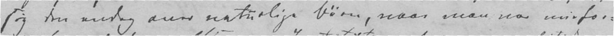sig den endog over naturlige Børn, naar man var misfor-
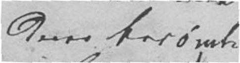deres berømte
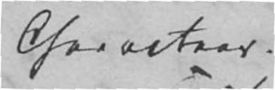Characteer.
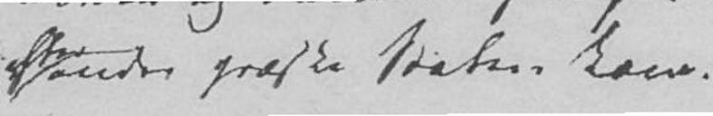kender græske Staters Love.
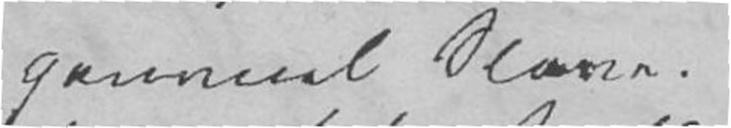gammel Slave.
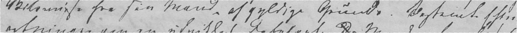Skilsmisse fra sin Mand af gyldige Grunde. Bestemte Efter-
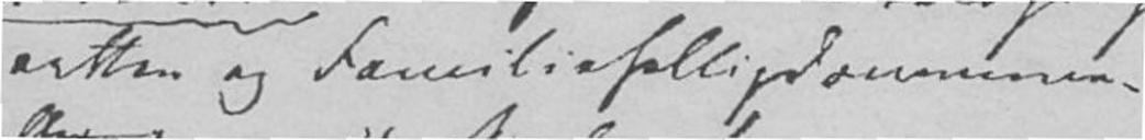retten og Familiehelligdommene.
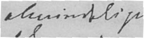almindelige
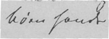Børn havde
